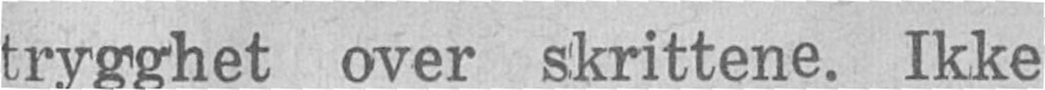trygghet over skrittene. Ikke
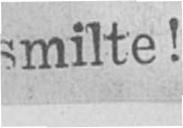smilte!
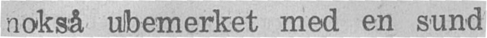nokså ubemerket med en sund
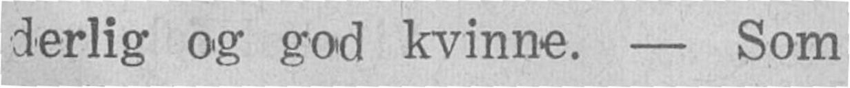derlig og god kvinne. - Som
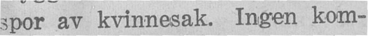spor av kvinnesak. Ingen kom-
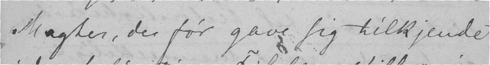Magter, der før gave sig tilkjende
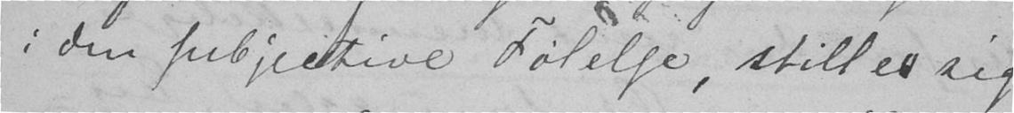i den subjective Følelse, stiller sig
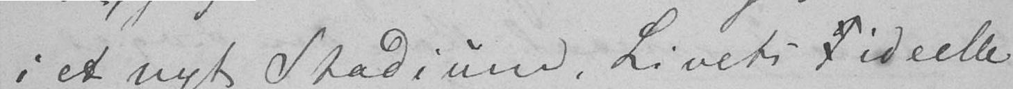i et nyt Stadium. Livets T ideelle
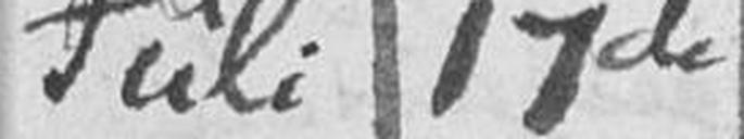Juli 17de
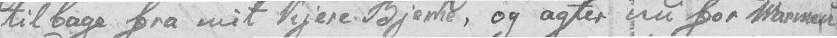tilbage fra mit kjere Bjerke, og agter nu for Varmen
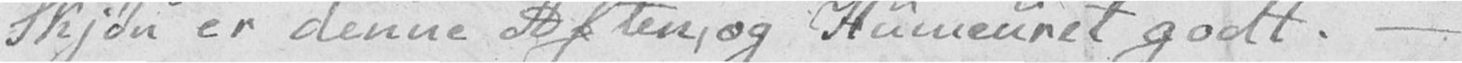Skjøn er denne Aften, og Humeuret godt. –
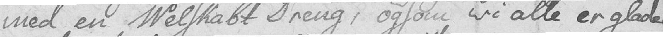med en Velskabt Dreng, og som vi alle er glade
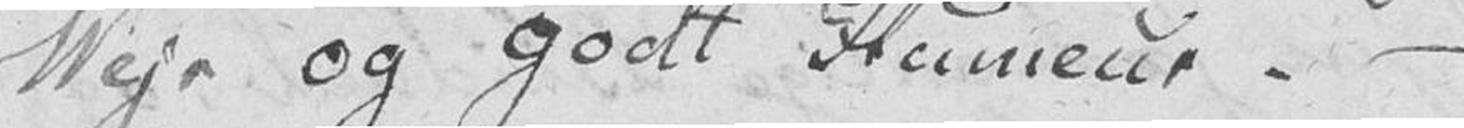Vejr og godt Humeur. –
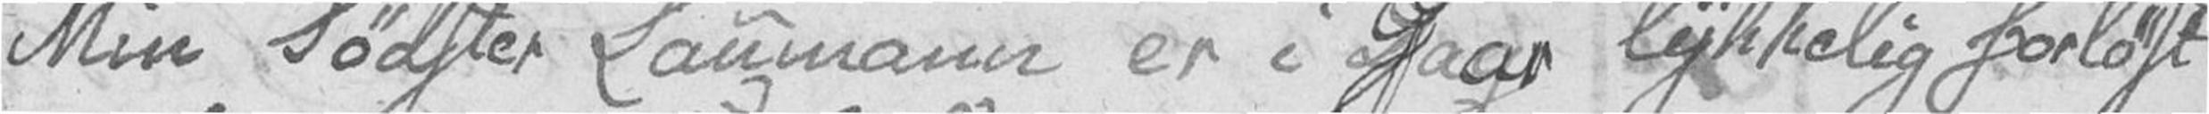Min Sødster Laumann er i Gaar lykkelig forløst
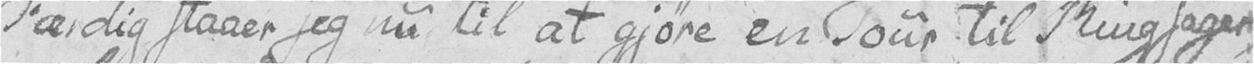Færdig staaer jeg nu til at gjøre en Tour til Ringsager,
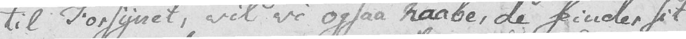til Forsynet, vil vi ogsaa haabe, de finder sit
